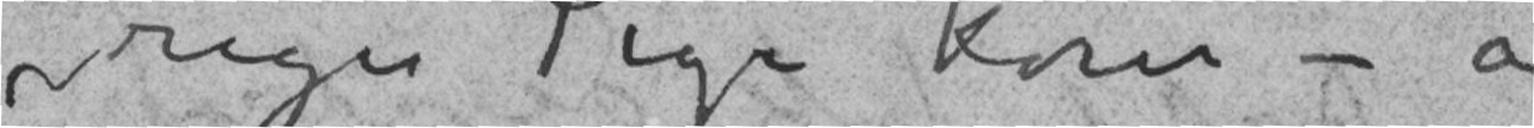rige Pige kom – og
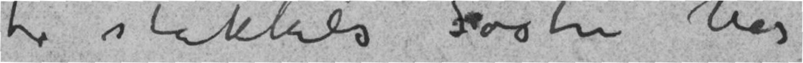to stakkels Søstre har
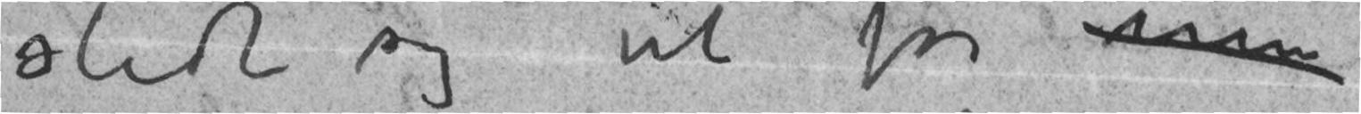slidt sig ud for min
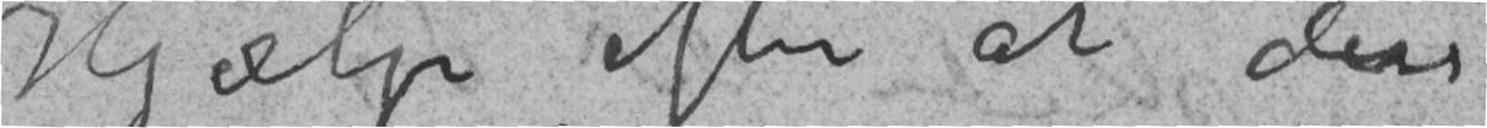Hjælp efter at den
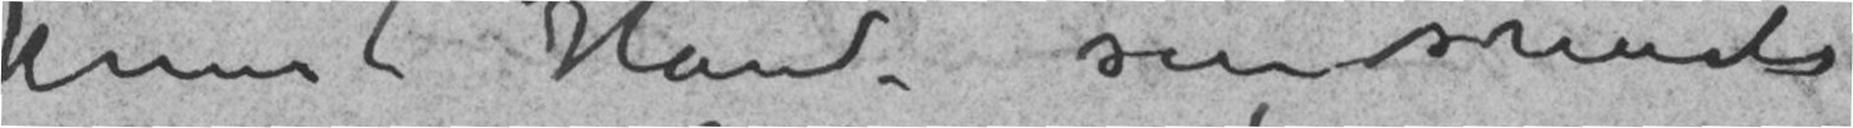knust Hånd som smerter
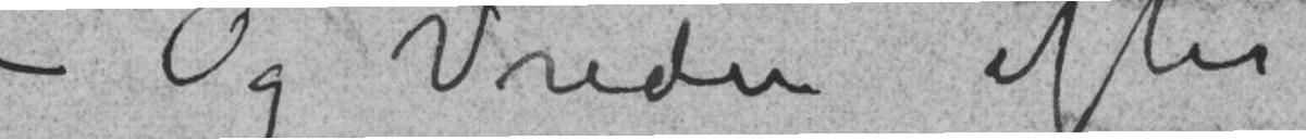– Og Vreden efter
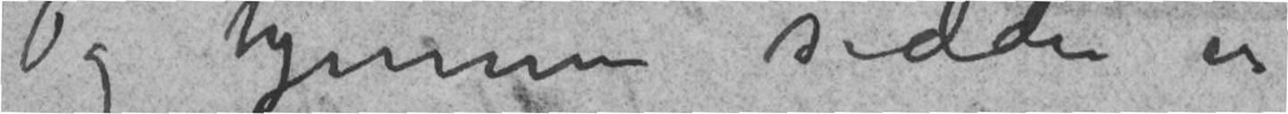Og hjemme sidder en
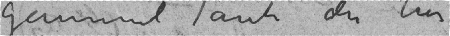gammel Tante der har
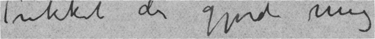Trekket der gjorde mig
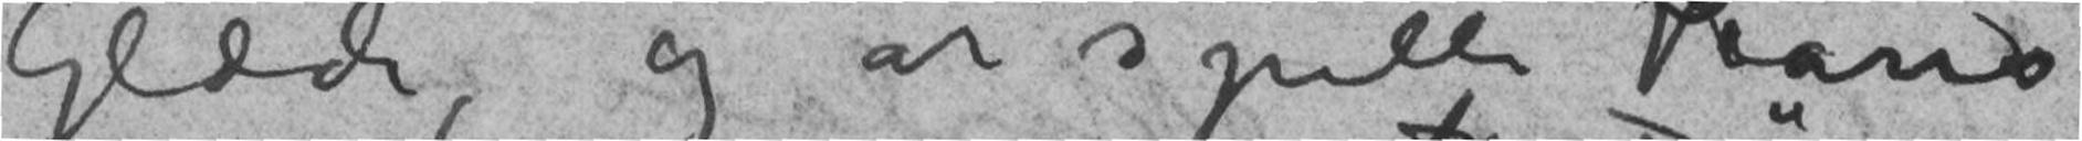Glæde, og at spille Piano
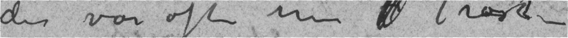der var ofte minTrøst –
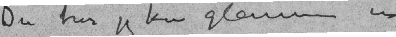Du tror jeg kan glemme en
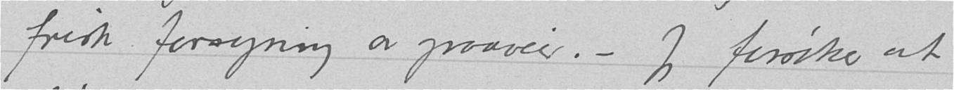frisk forsyning av graaveir. – Jeg forsøker at
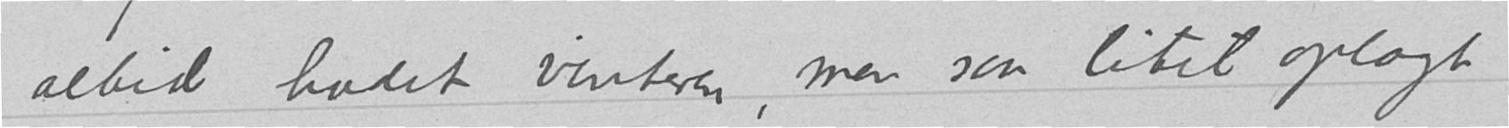altid hadet vinteren, men saa litet oplagt
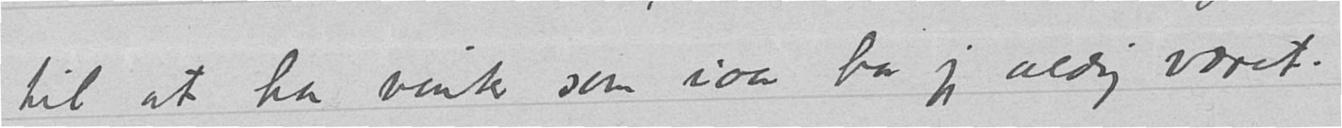til at ha vinter som iaar har jeg aldrig været.
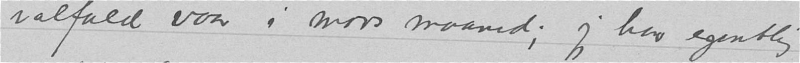ialfald vaar i mars maaned; jeg har egentlig
